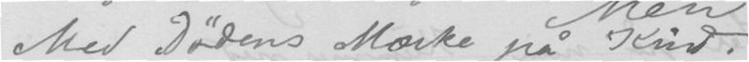Med Dødens Mærke på Kind.
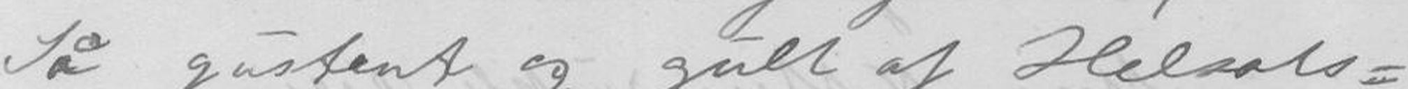Så gustent og gult af Helsats-
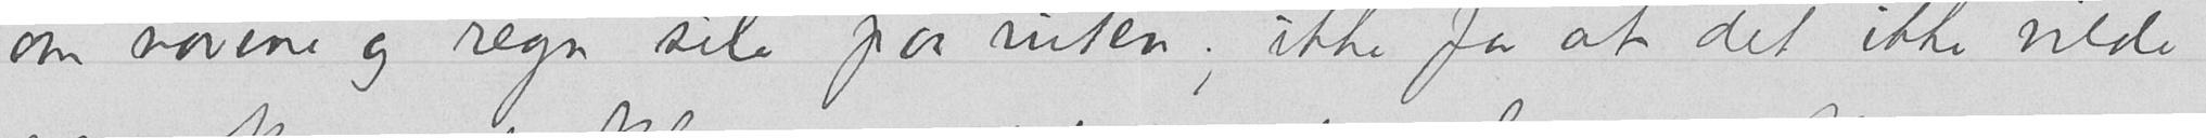om novene og regn sile paa ruten; ikke for at det ikke vilde
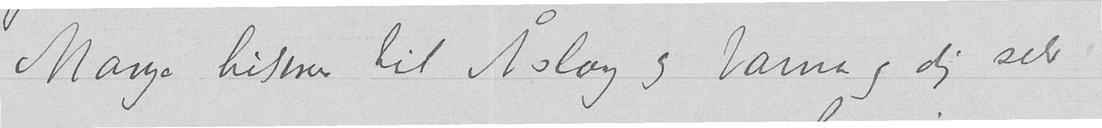Mange hilsener til Åslaug og barna og dig selv
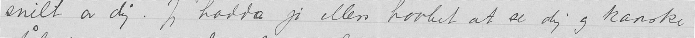snilt av dig. Jeg hadde jo ellers haabet at se dig og kanske
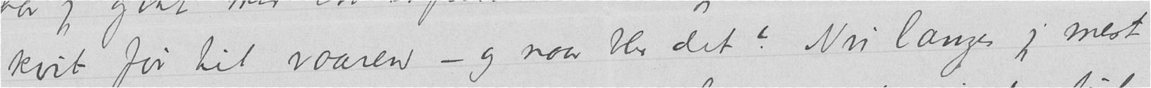kvit før til vaaren – og naar blir det? Nu længes jeg mest
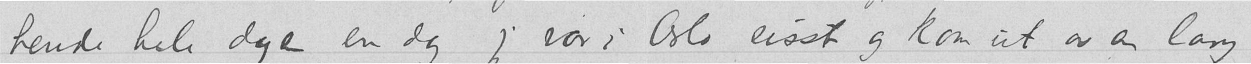hende hele dagen en dag jeg var i Oslo sisst og kom ut av en lang
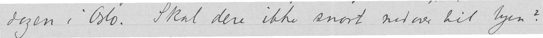dagen i Oslo. Skal dere ikke snart nedover til byen?
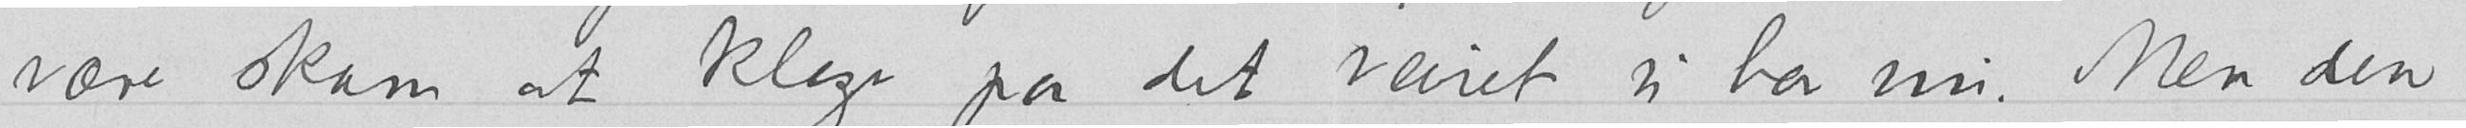være skam at klage paa det veiret vi har nu. Men den
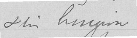Din hengivne
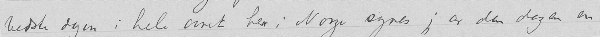bedste dagen i hele aaret her i Norge synes jeg er den dagen en
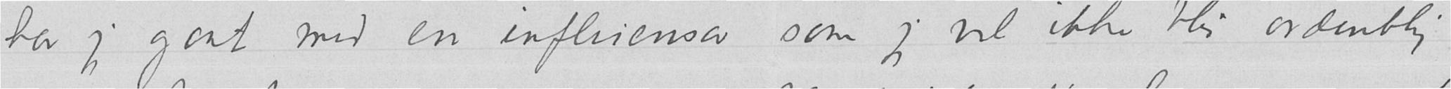har jeg gaat med en influensa som jeg vel ikke blir ordentlig
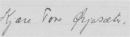Kjære Tore Øjasæter,
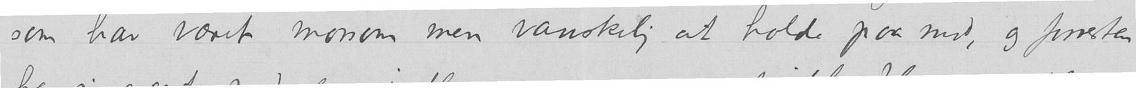som har været morsom men vanskelig at holde paa med, og forresten
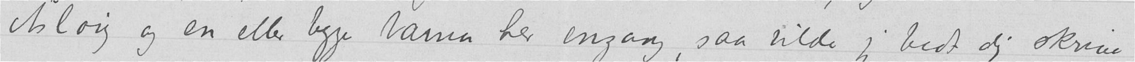Åslaug og en eller begge barna her engang, saa vilde jeg bedt dig skrive
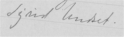Sigrid Undset.
